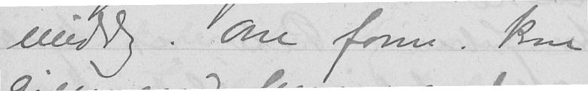middag. Om form. kom
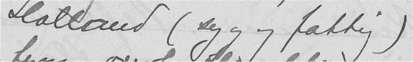Halland (syg og fattig)
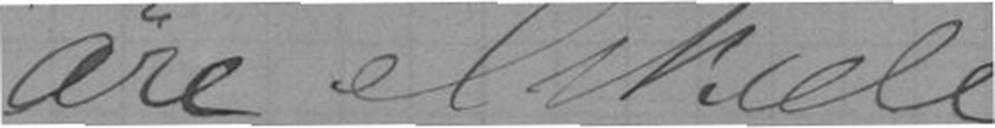Kjære elskede
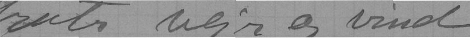trots vejr og vind
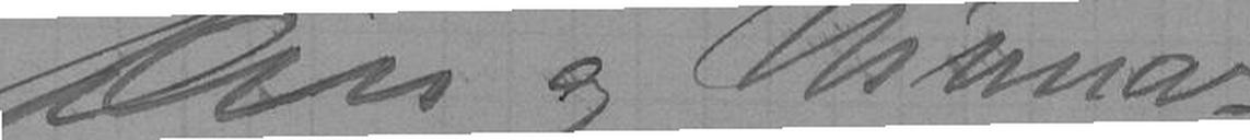Din og Ninnas
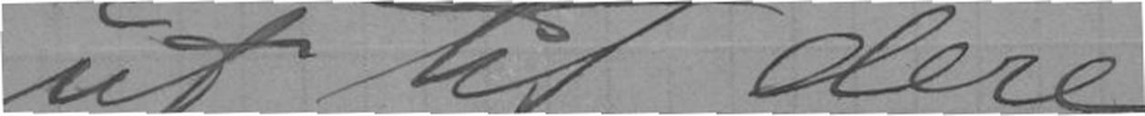ut til dere
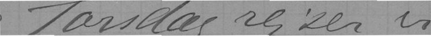6 Torsdag rejser vi
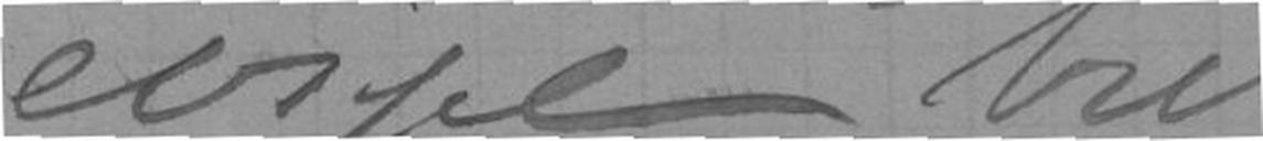evige tro.
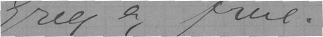Grieg og Frue!
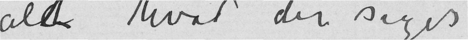allt hvad der siges
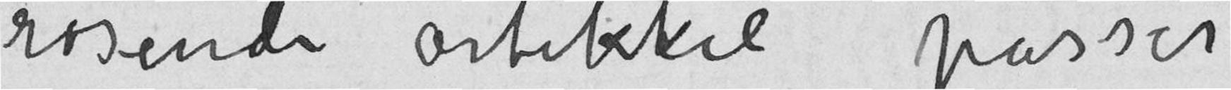rosende artikkel passer
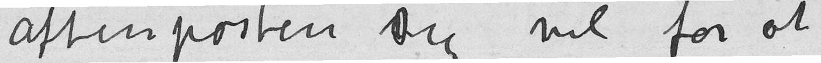Aftenposten sig vel for at
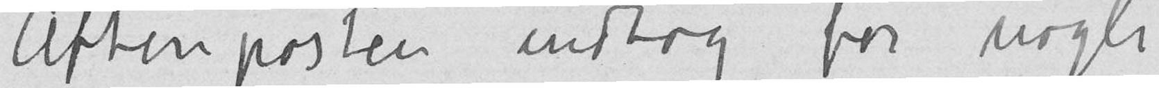Aftenposten indtog for nogle
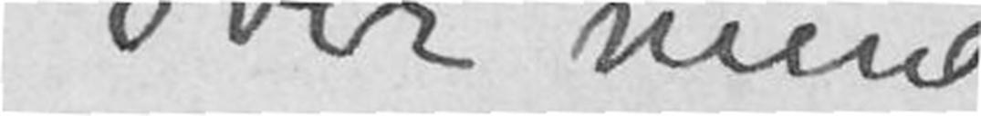over mine
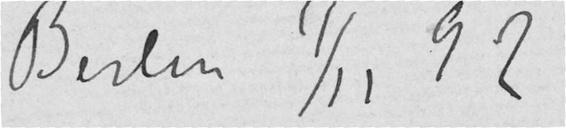Berlin 11/11 92
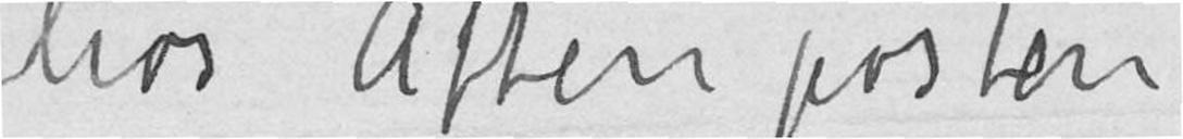hos Aftenposten
